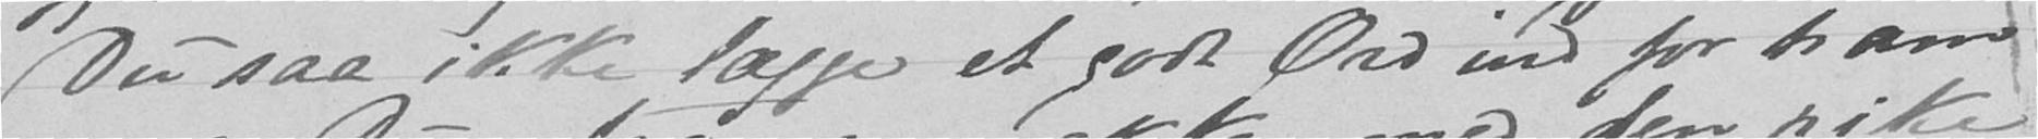Du saa ikke lægge et godt Ord ind for ham
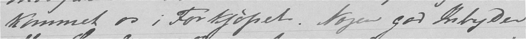kommet os i Forkjøpet. Nogen god Inbyder
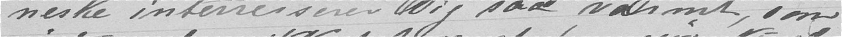neske interessere Dig saa varmt, som
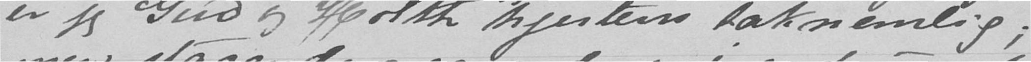er jeg Gud og Holth hjertens taknemlig;
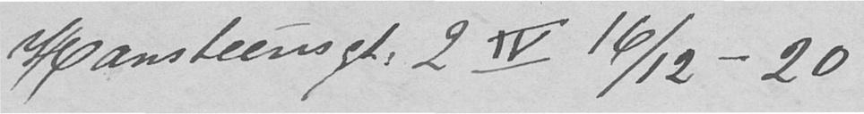Hansteensgt. 2 IV 16/12 - 20
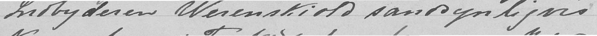Indbyderen Werenskiold sandsynligvis
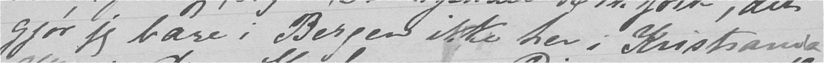gjør jeg bare i Bergen ikke her i Kristiania.
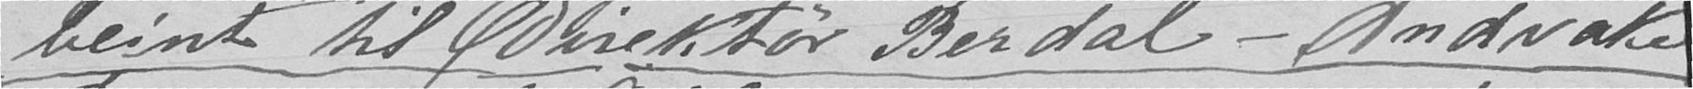"beint til Direktør Berdal - Andvake
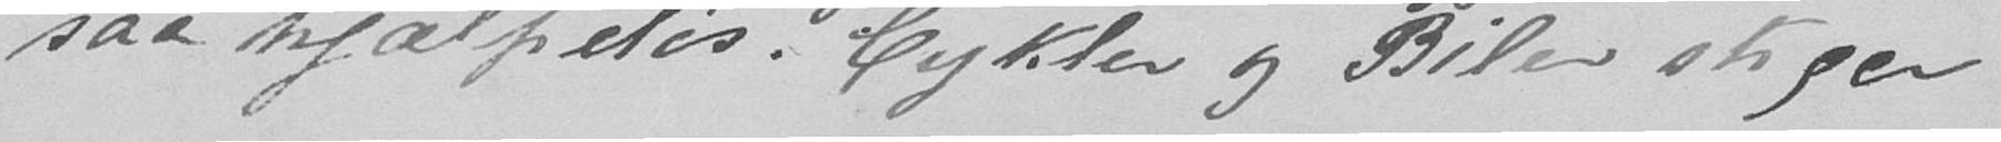saa hjælpeles. Cykler og Biler stiger
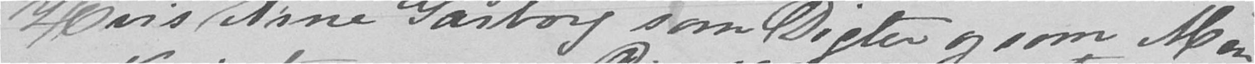Hvis Arne Garborg som Digter og som Men-
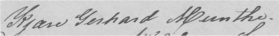Kjære Gerhard Munthe.
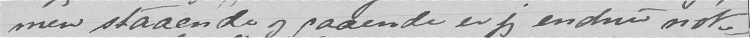men staaende og gaaende er jeg endnu nok-
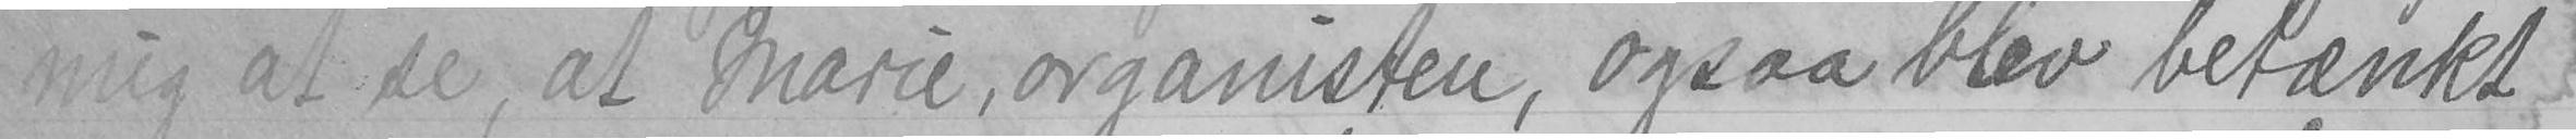mig at se, at Marie, organisten, ogsaa blev betænkt
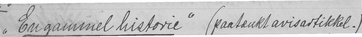"En gammel historie" (paatænkt avisartikkel.)
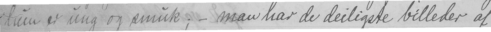hun er ung og smuk; - man har de deligste billeder af
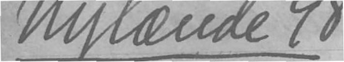Nylænde 98
